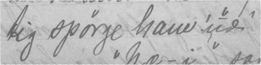tig spørge ham! ud"
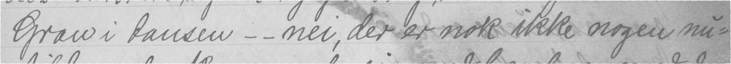Gran i dansen - - nei, der er nok ikke nogen nu-
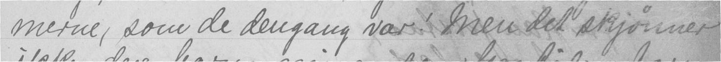merne, som de dengang var! Men det skjønner
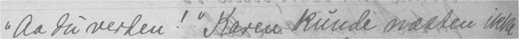"Aa du verden!" Karen kunde næsten ikke
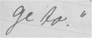ge to."
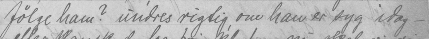følge ham? undres rigtig, om han er syg idag -
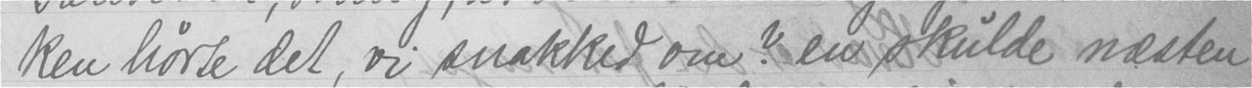ken hørte det, vi snakked om? en skulde næsten
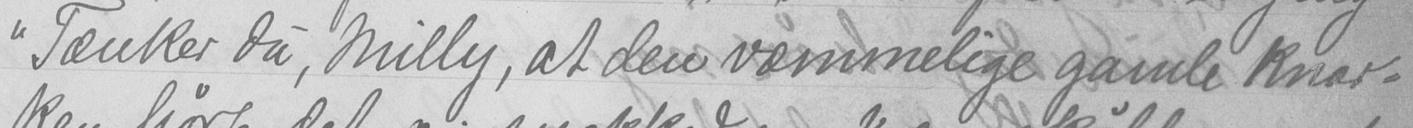"Tænker du, Milly, at den væmmelige gamle knar-
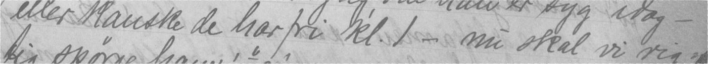eller kanske de har fri kl. 1 - nu skal vi rig-
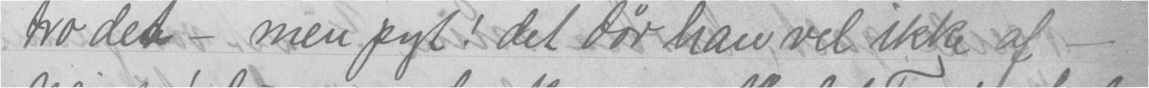tro det - men pyt! det dør han vel ikke af -
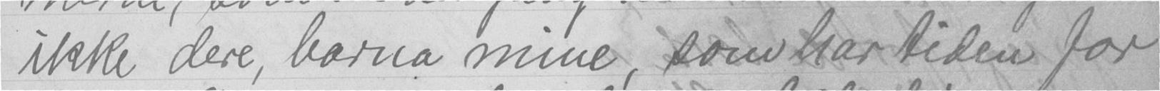ikke dere, barna mine, som har tiden for
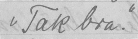"Tak bra."
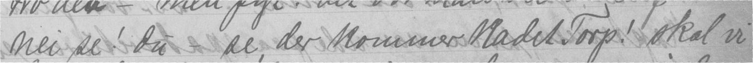nei se! du - se, der kommer kadet Torp! skal vi
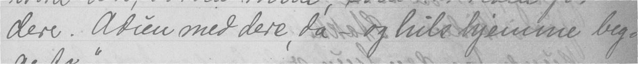dere. Adieu med dere, da - og hils hjemme beg-
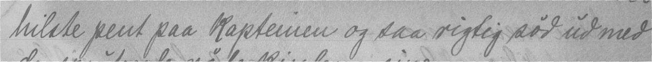hilste pent paa kapteinen og saa rigtig sød ud med
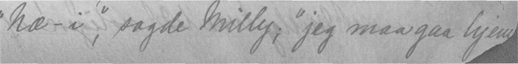"Næ-i", sagde Milly; "jeg maa gaa hjem
